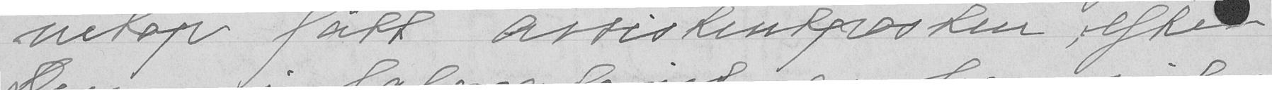netop fått assistentposten efter
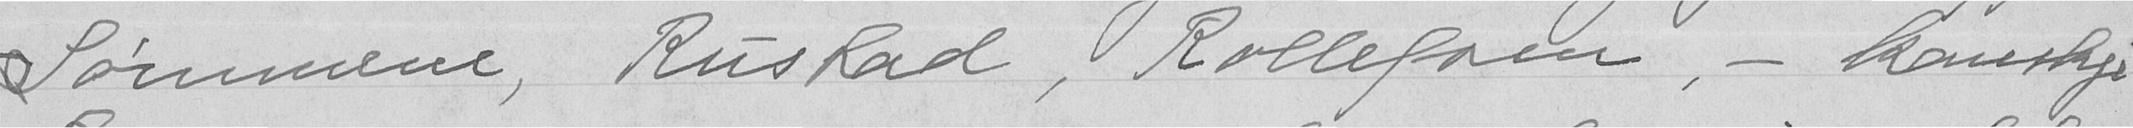Sømmene, Rustad, Rollegaen - kanskje
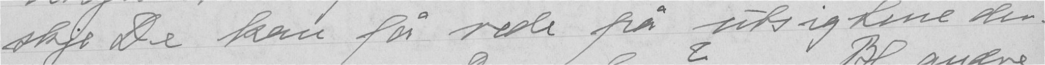skje De kan få rede på utsigtene der-
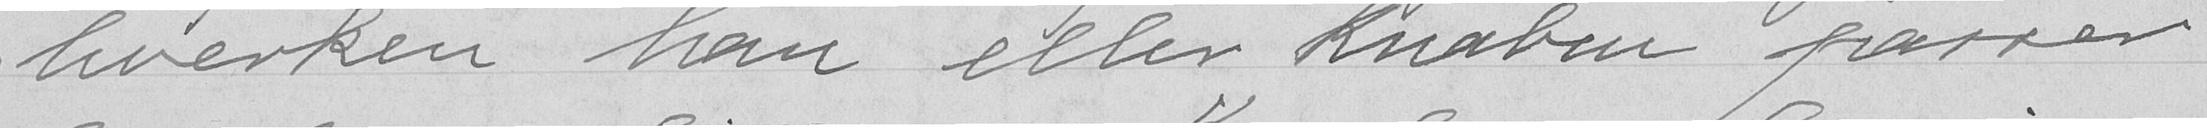hverken han eller Knaben passer
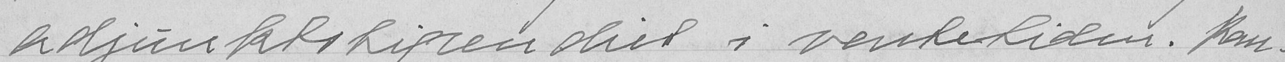adjunktstipendiet i ventetiden. Kan-
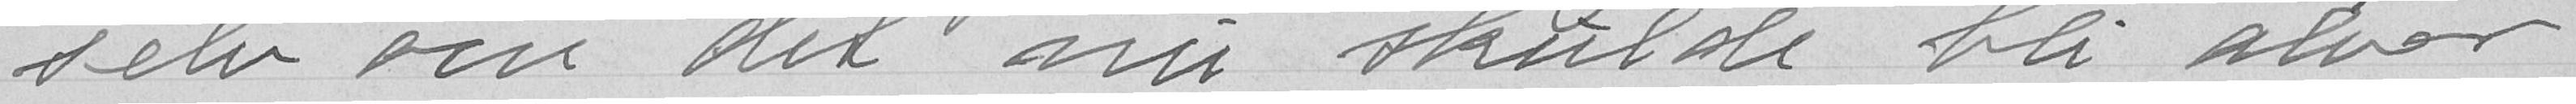selv om det nu skulde bli alvor
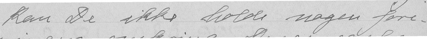Kan De ikke holde nogen fore-
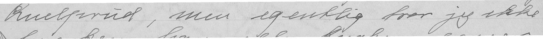Ruelprud, men egentlig tror jeg ikke
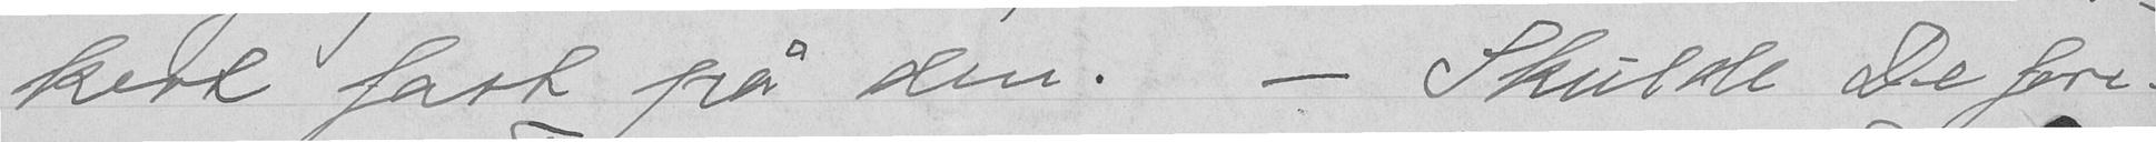kert fast på den. Skulde De fore-
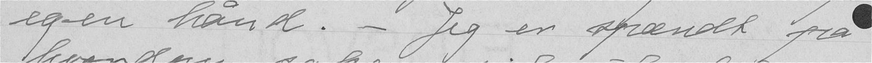egen hånd. - Jeg en spændt på
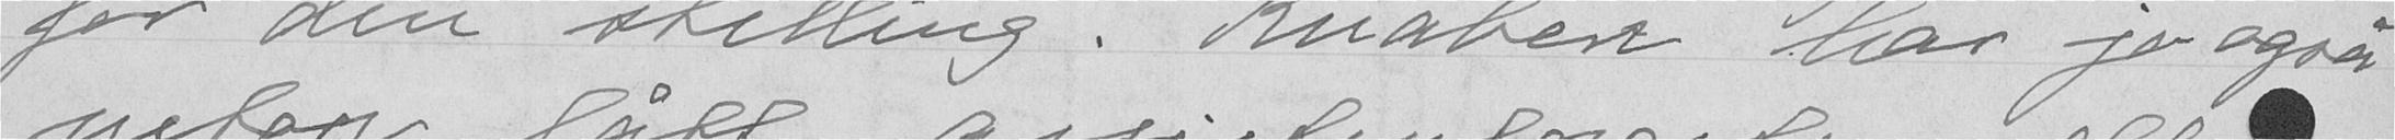for den stilling. Knaben har jo også
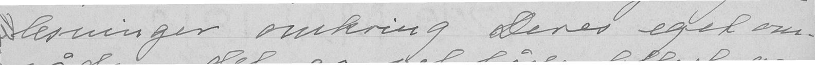lesninger omkring Deres eget om-
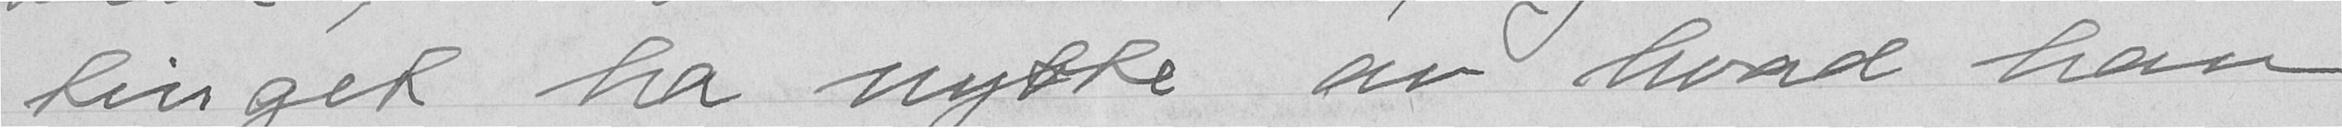tinget ha nytte åv hvad han
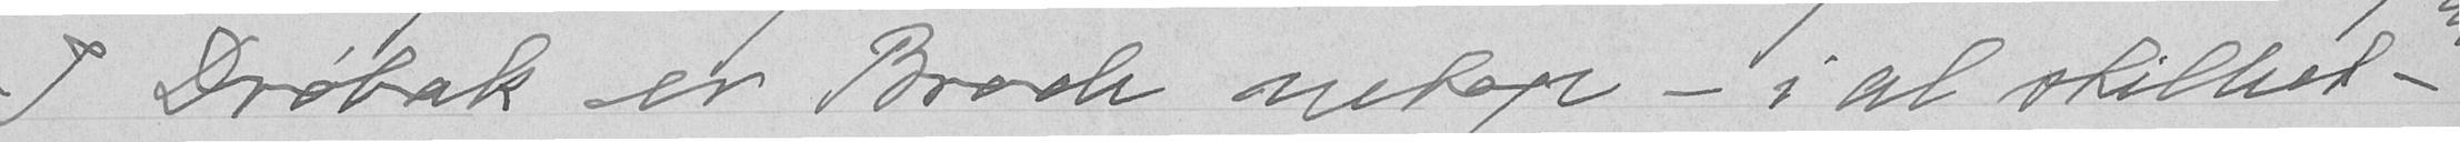I Drøbak er Broch netop - i at stilhet -
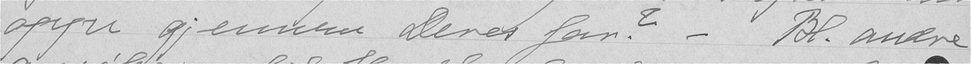oppe gjennem Deres far? - Bl. andre
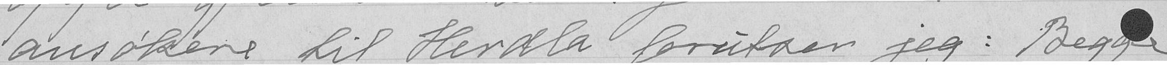ansøkere til Herdla forutser jeg: Begge
